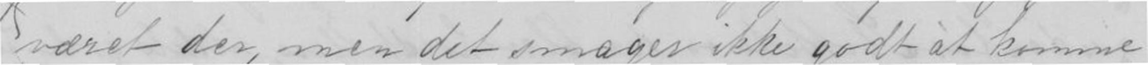været der, men det smager ikke godt at komme
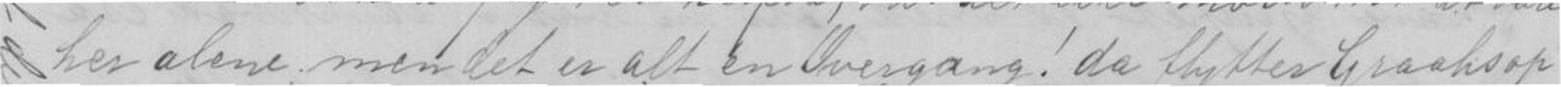her alene, men det er alt en Overgang! da flytter Graahsop
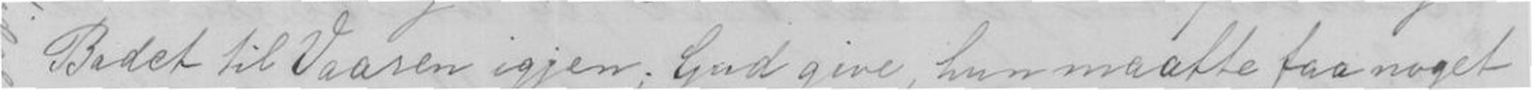Badet til Vaaren igjen; Gud give, hun maatte faa noget
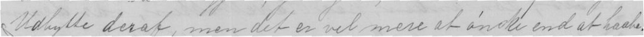Udbytte deraf, men det er vel mere at ønske end at Haabe.
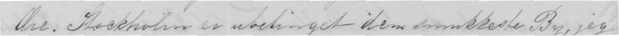Ære. Stockholm er ubetinget den smukkeste By, jeg
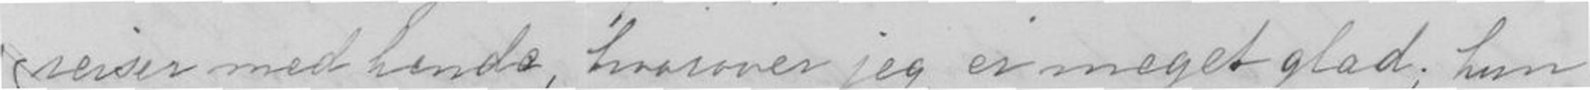reiser med hende, hvorover jeg er meget glad; hun
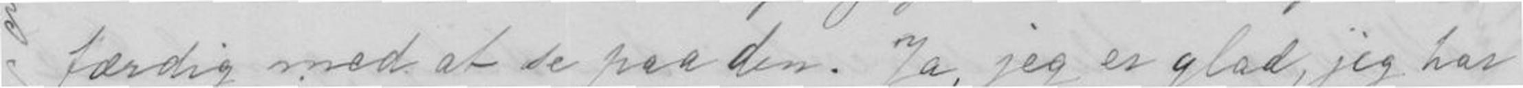færdig med at se paa den. Jo, jeg er glad, jeg har
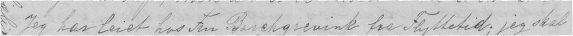Jeg har leiet hos Fru Barchgrevink fra Flyttetid; jeg skal
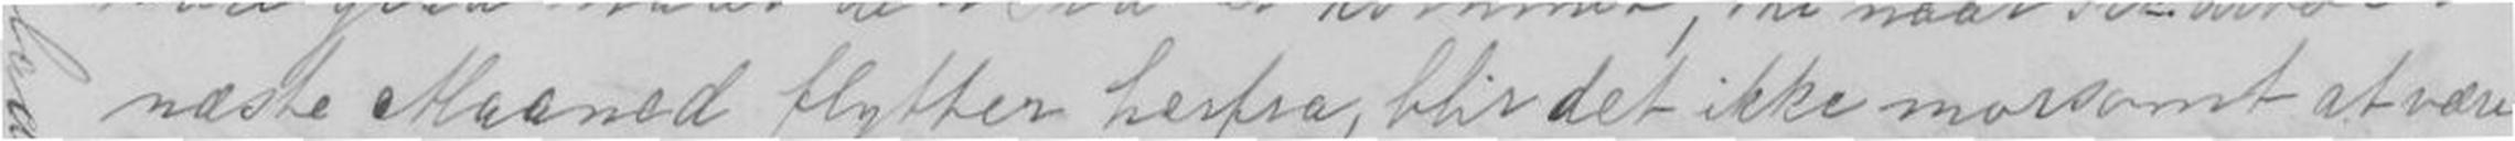næste Maaned flytter herfra, blir det ikke morssomt at være
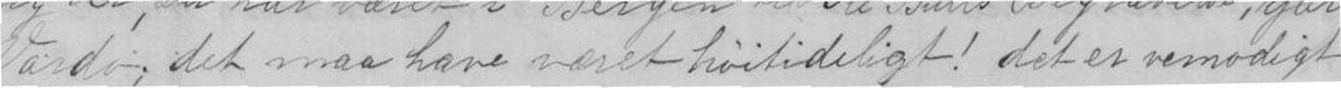Vardo,- det maa have været høitideligt! det er vemodigt
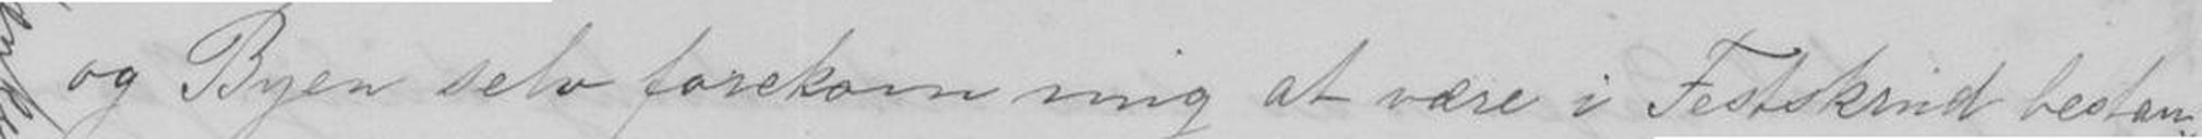og Byen selv forekom mig at være i Festskrud bestan-
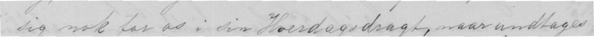sig nok for os i sin Hverdagsdragt, naar undtages
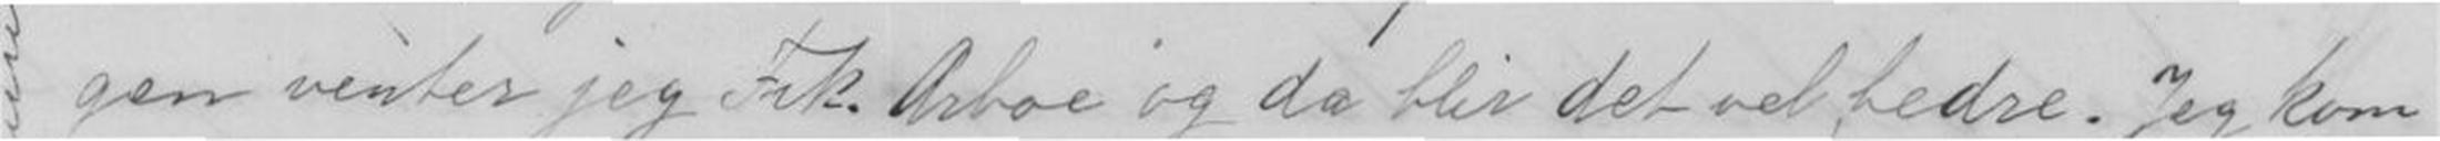gen venter jeg Frk.Arboe og da blir det vel, bedre. Jeg kom
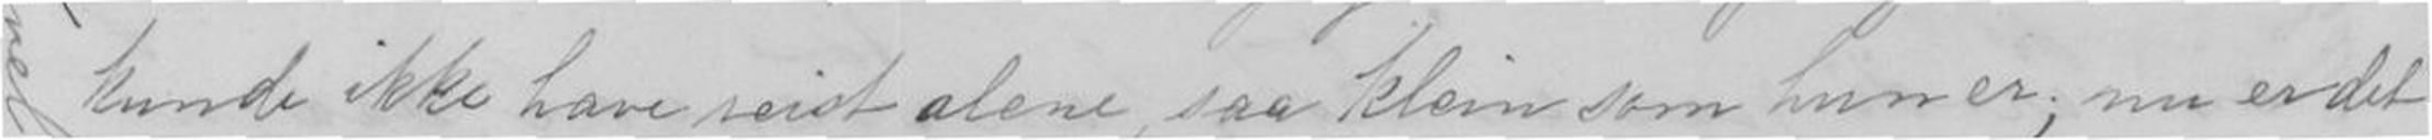kunde ikke have reist alene, saa klein som hun er; nu er det
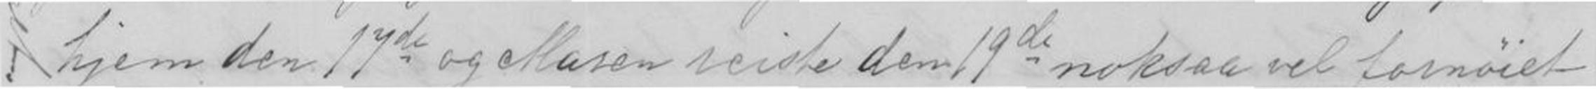hjem den 17de og Moren reiste den 19de noksaa vel fornøiet
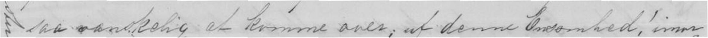saa vanskelig at komme over; uf denne Ensomhed! imor-
